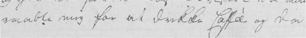raabte mig for at drikke Caffee og da
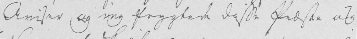Aviser og jeg frygtede disse Præste og
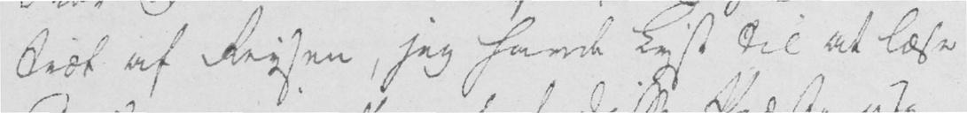træt af Reysen, jeg havde Lyst til at læse
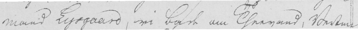mand Lysgaard, vi bade om Theevand, Verten
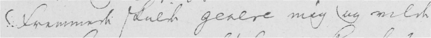fremmede skulde genere mig og vilde
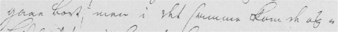gaae bort, men i det samme kom de og i
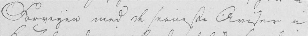Forveyen med de seeneste Aviser i
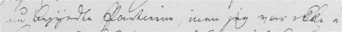nu begyndte Partierne, men jeg var ikke i
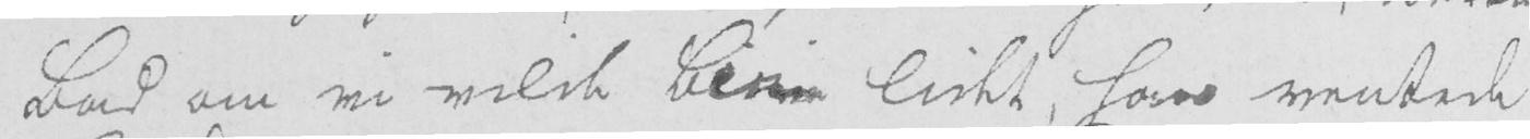bad om vi vilde blive lidt, han ventede
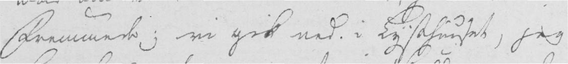Fremmede; vi gik ned i Lysthuuset, jeg
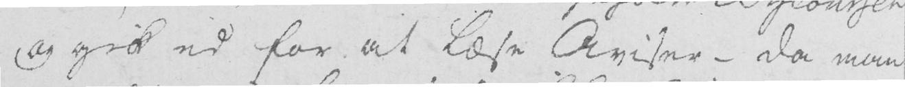og gik ud for at læse Aviser - da man
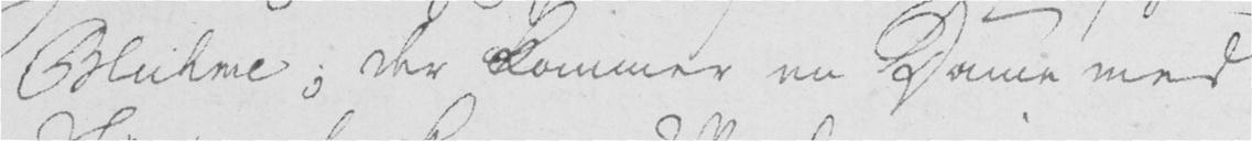Bluhme; Der kommer en Dame med
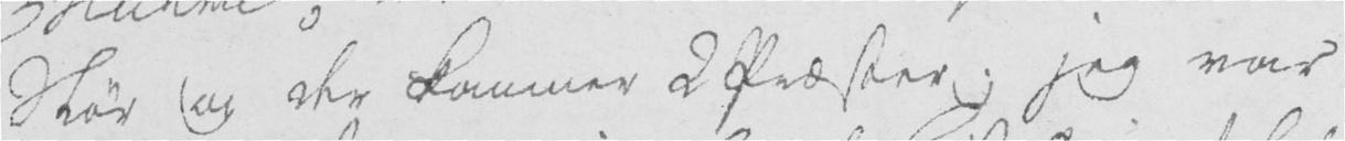Skør og der kommer 2 Præster; jeg var
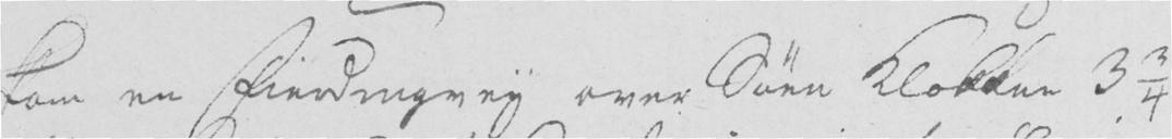kom en Fierdingvey over Søen Klokken 3 3/4
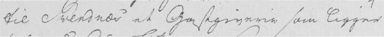til Svendnæs et Gastgiverie som ligger
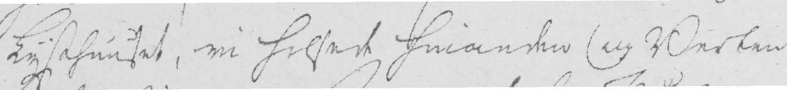Lysthuuset, vi hilsede hinanden og Verten
